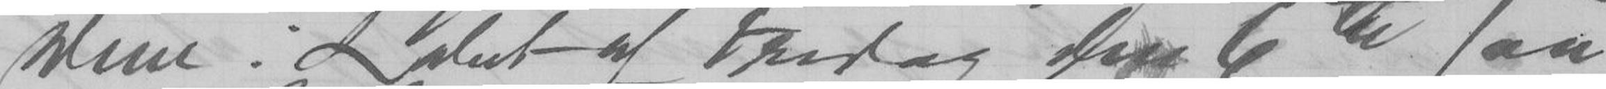Dem i løbet af Fredag dan 6te saa
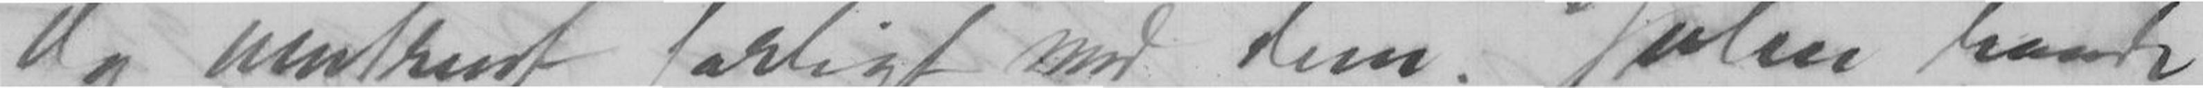da omtrent færdigt med den. John havde
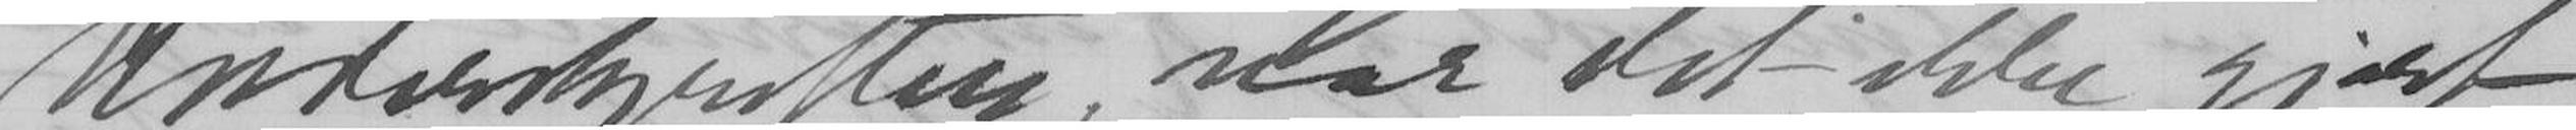Underskriften, var det ikke gjort.
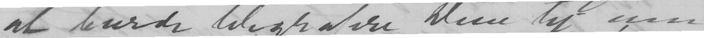at burde telegrafere Dem til om
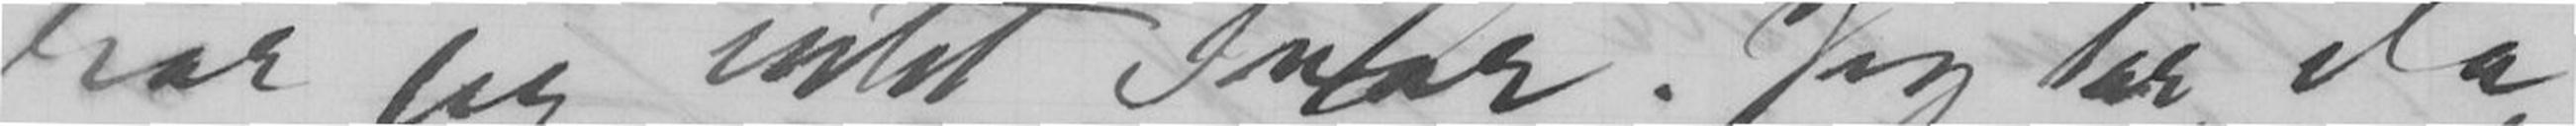har jeg intet Svar. Jeg tør da
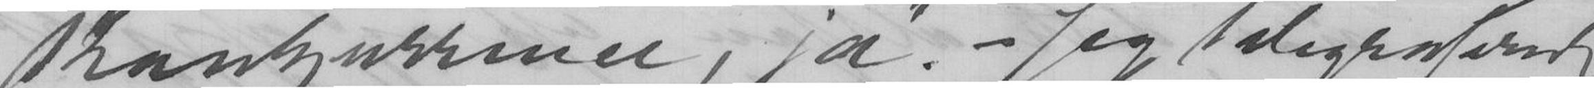Konkurrance, ja. - Jeg telegraferede
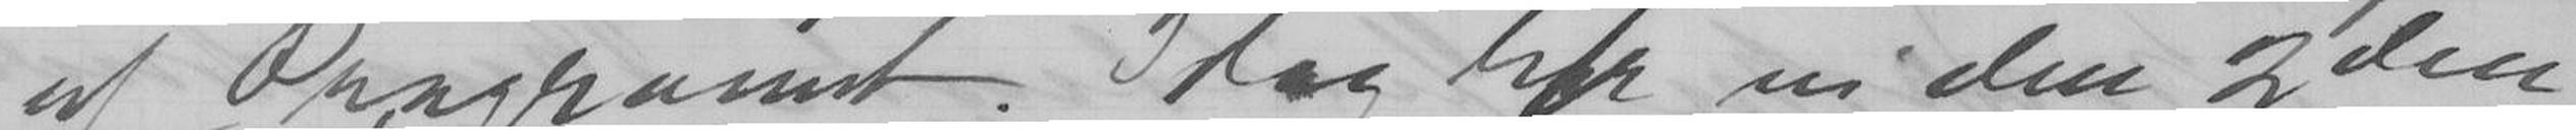af Programet. Idag har vi den 2den.
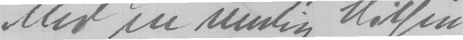Med en venlig Hilsen
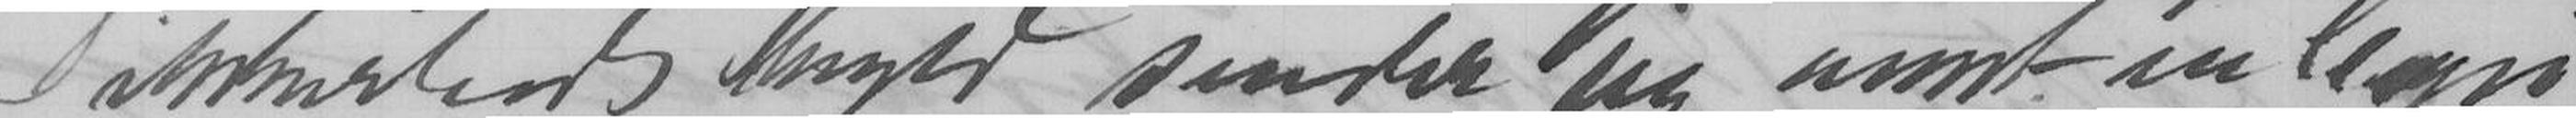Sikkerheds Skyld sender jeg samt en Copi
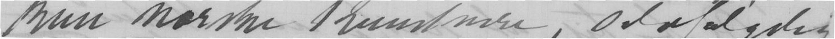kun norske Kunstnere, selvfølgelig
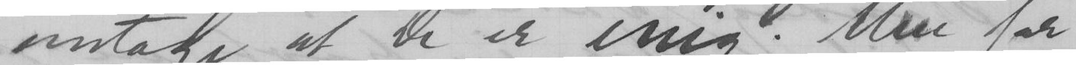antage at De er enig. Men for
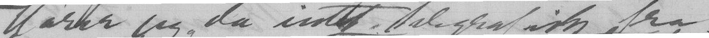Hører jeg da intet telegrafisk fra
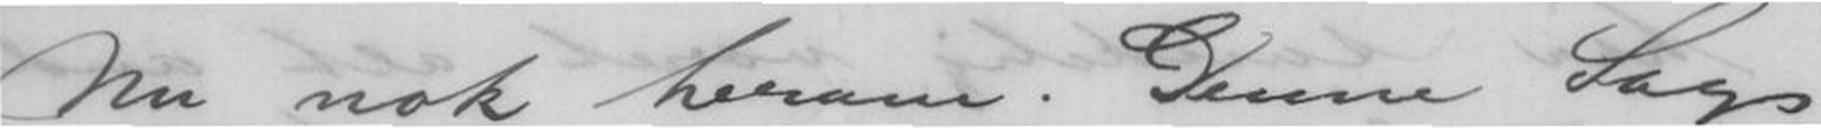Nu nok herom. Denne Sag
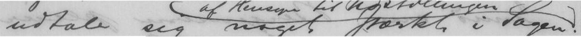udtale sig noget stærkt i Sagen.
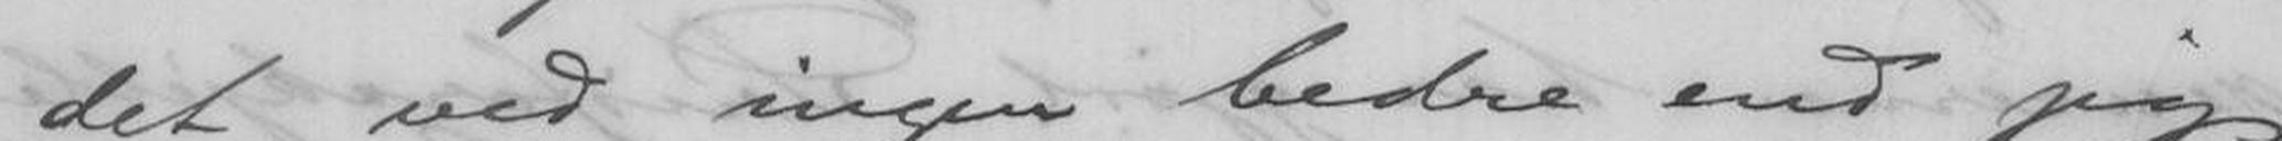et ved ingen bedre end jeg,
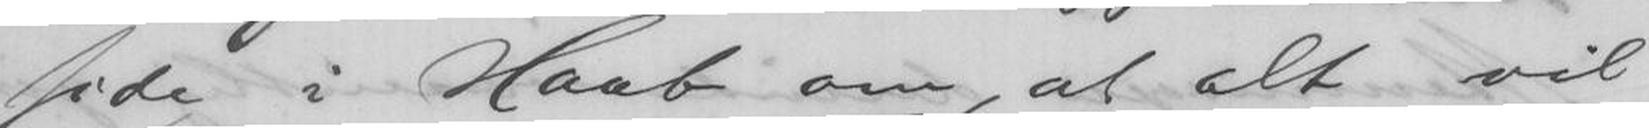side i Haab om, at alt vil
